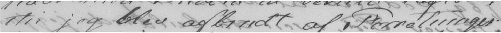Thi jeg blev afbrudt af Forretninger
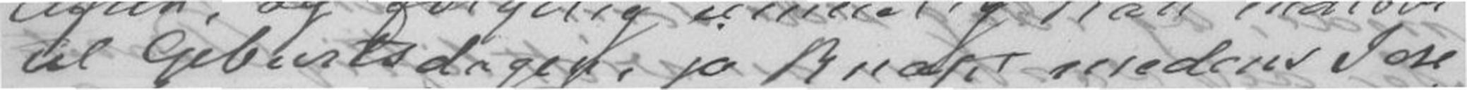til Geburtsdagen; jo knapt medens I ere
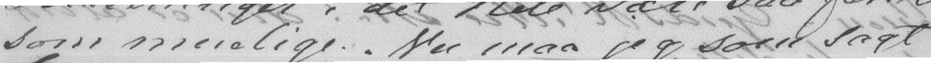som muelig. Nu maa jeg som sagt
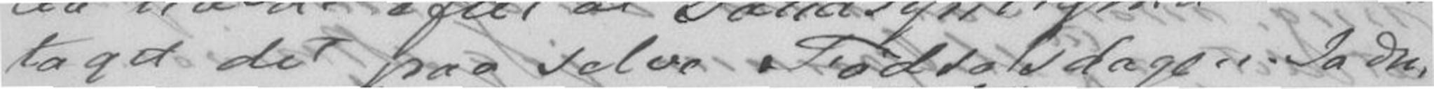taget det paa selve Fødselsdagen. Ja du,
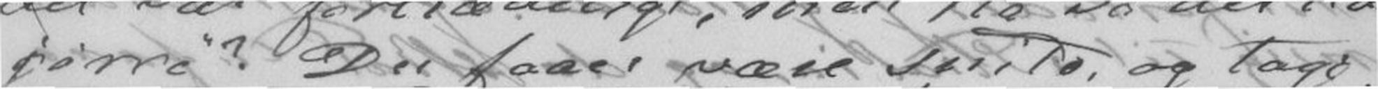jørre"? Du faaer være snild og tage
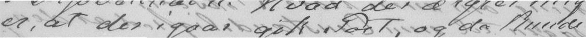er, at der igaar gik Post, og da kunde
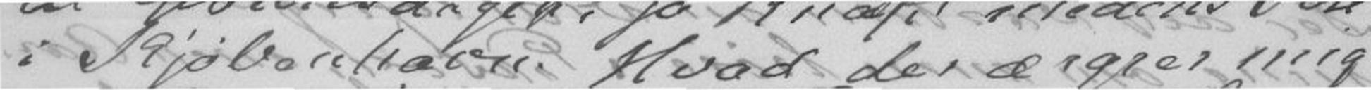i Kjøbenhavn. Hvad der ærgrer mig
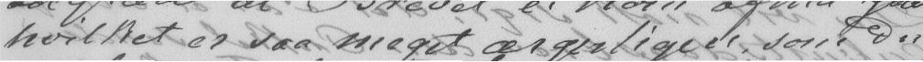hvilket er saa meget ærgerligere, som Du
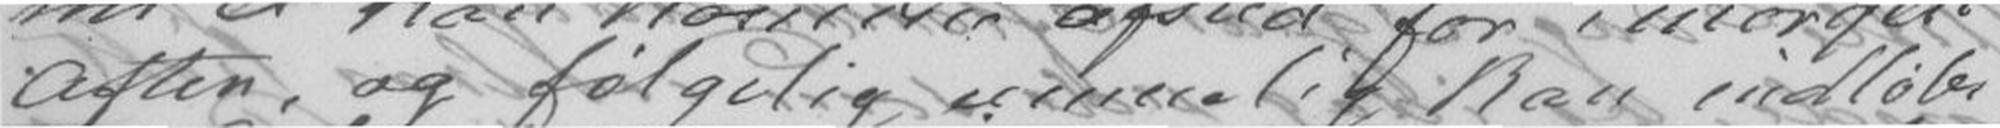Aften, og følgelig umuelig kan indløbe
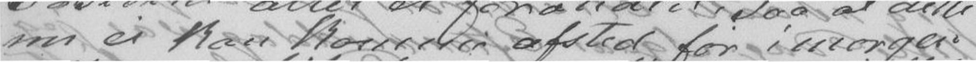nu ei kan komme afsted for i morgen
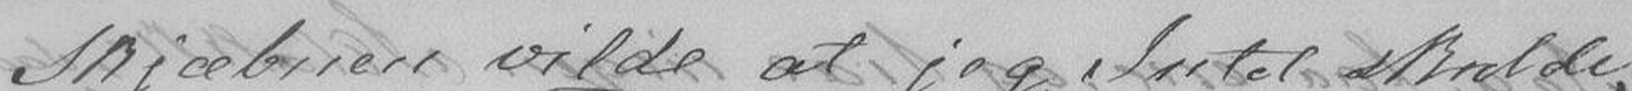Skjæbnen vilde at jeg Intet skulde
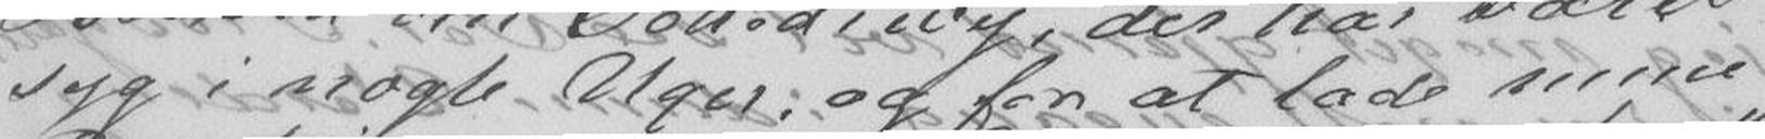syg i nogle Uger, og for at lade mine
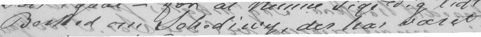Besked om Jchediwy, der har været
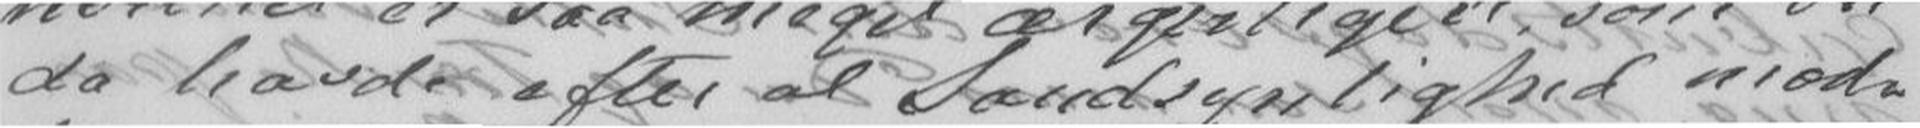da havde efter al Sandsynlighed mod-
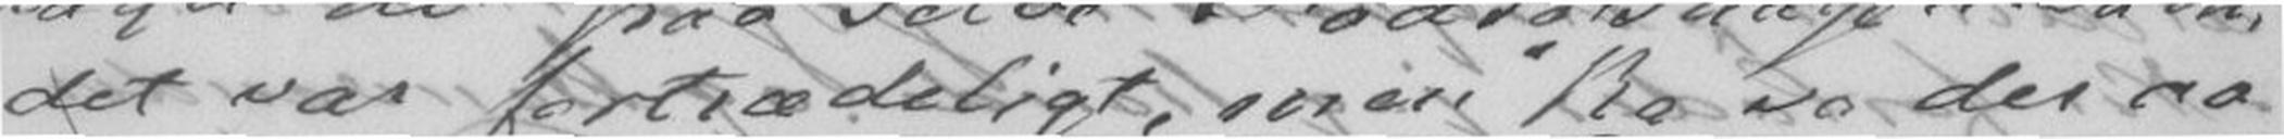det var fortrædeligt, men "ka va der aa
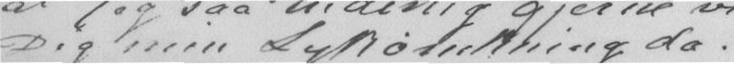Dig min Lykønskning da.
